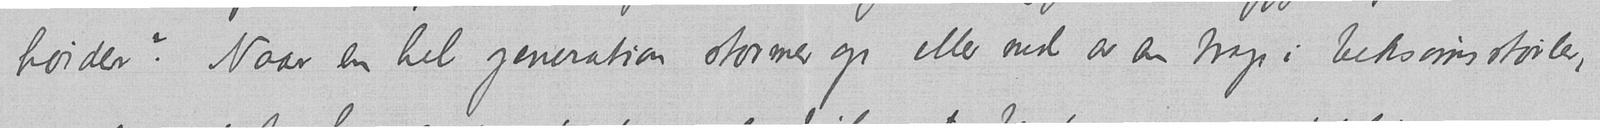høider? Naar en hel generation stormer op eller ned av en trap i beksømsstøvler,
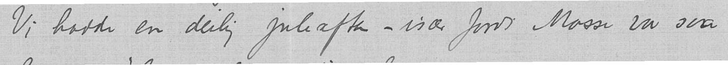Vi hadde en deilig juleaften – især fordi Mosse var saa
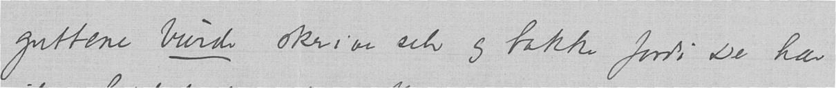guttene burde skrive selv og takke fordi De har
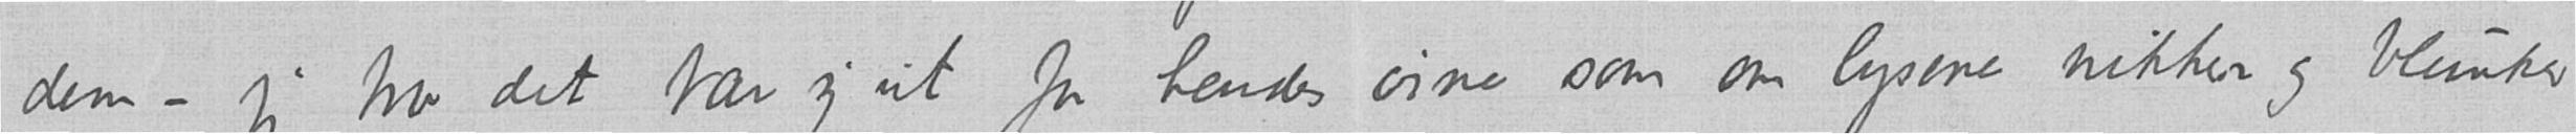dem – jeg tror det tar seg ut for hendes øine som om lysene nikker og blunker
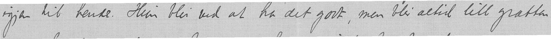igjen til hende. Hun blir ved at ha det godt, men blir altid litt grætten
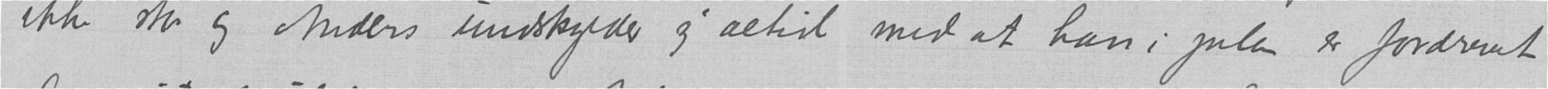ikke stor og Anders undskylder sig altid med at han i julen er fordrevet
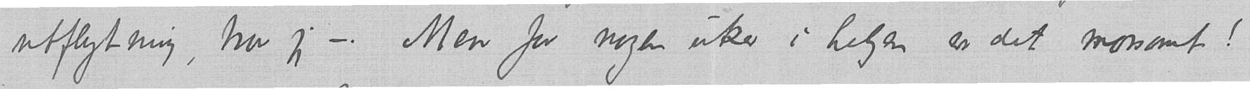utflytning, tror jeg –. Men for nogen uker i helgen er det morsomt!
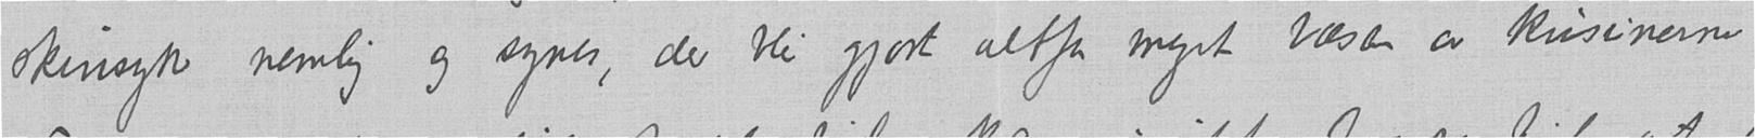skinsyk nemlig og synes, der blir gjort altfor meget væsen av kusinerne.
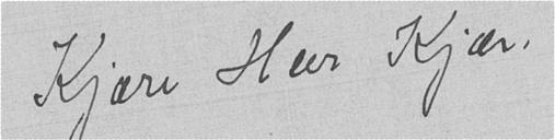Kjære Herr Kjær,
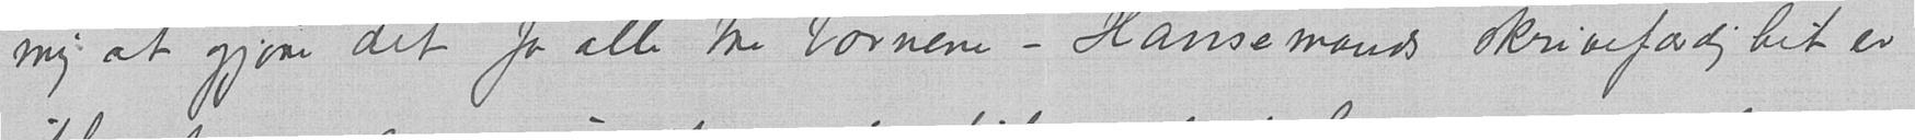mig at gjøre det for alle tre børnene - Hansemands skrivefærdighet er
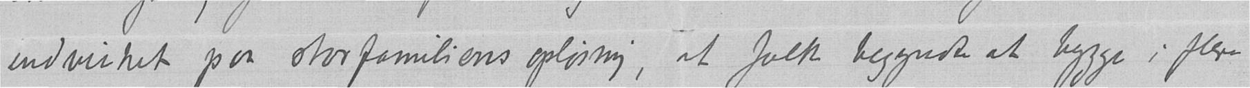indvirket paa storfamiliens opløsning, at folk begyndte at bygge i flere
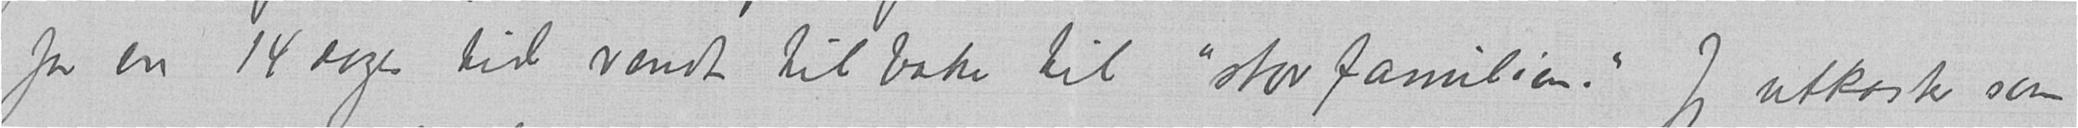for en 14 dages tid vændt tilbake til "storfamilien." Jeg utkaster som
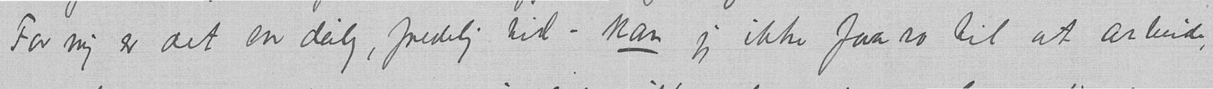For mig er det en deilig, fredelig tid – kan jeg ikke faa ro til at arbeide,
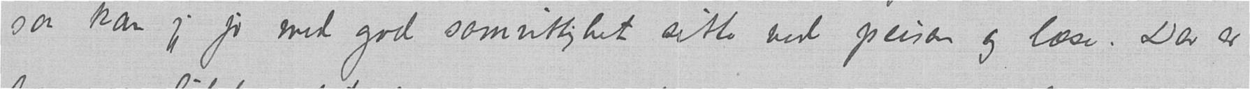saa kan jeg jo med god samvittighet sitte ved peisen og læse. Der er
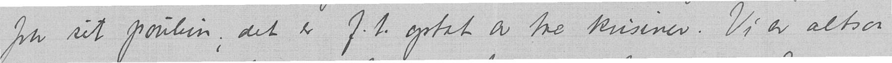fra sit poulun; det er f.t. optat av tre kusiner. Vi er altsaa
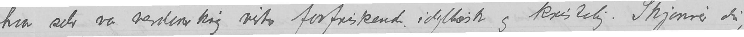hvor selv vor verdenskrig <virker> forfriskende idyllisk og kristelig.  Skjønner du,
